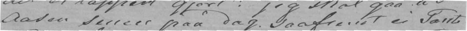Aasen senere paa Dag saafremt ei Tante
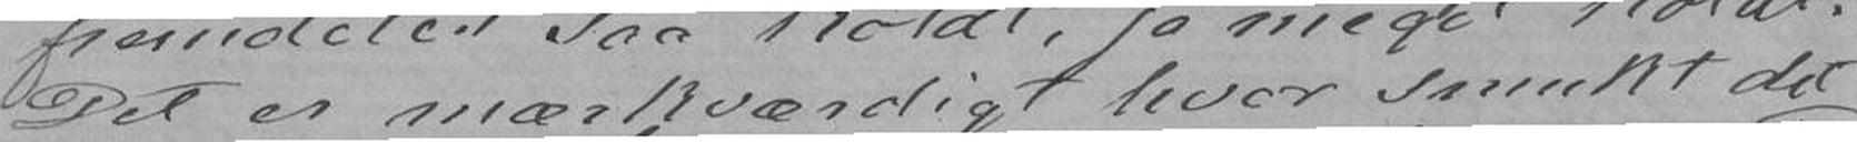Det er mærkværdigt hvor smukt det
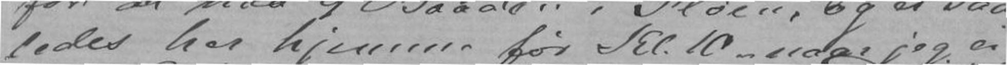ledes her hjemme før Kl.10 naar jeg ei
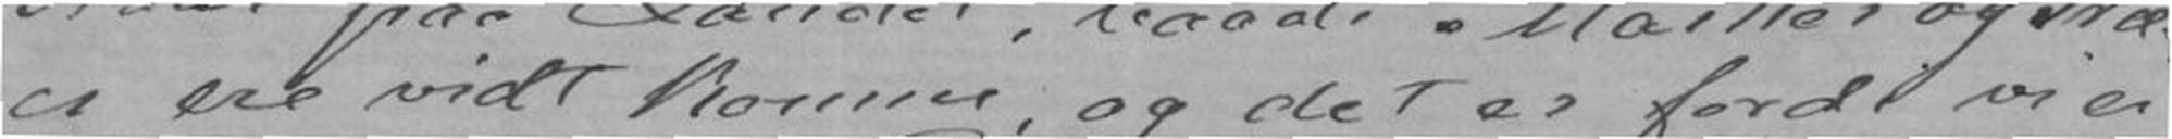er ere vidt komne, og det er fordi vi ei
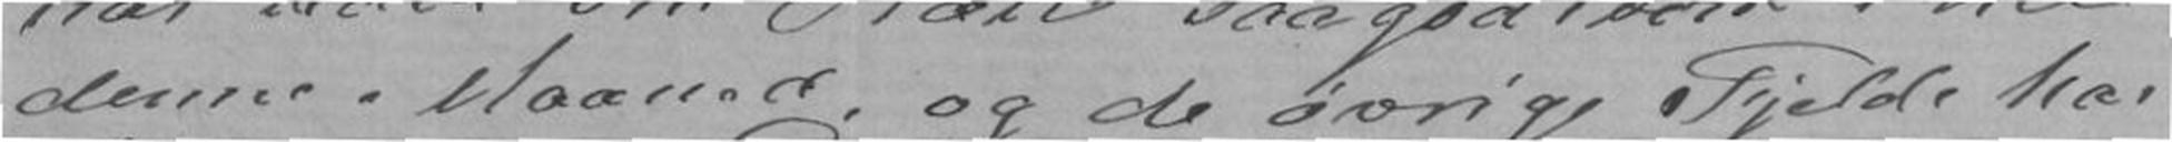denne Maned, og de øvrige Fjelde har
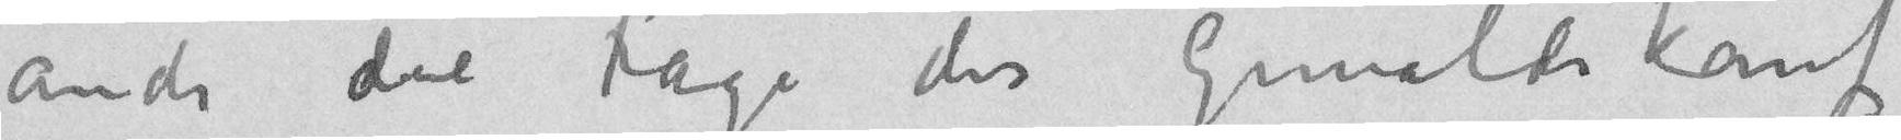auch die Frage des Gemaldekauf
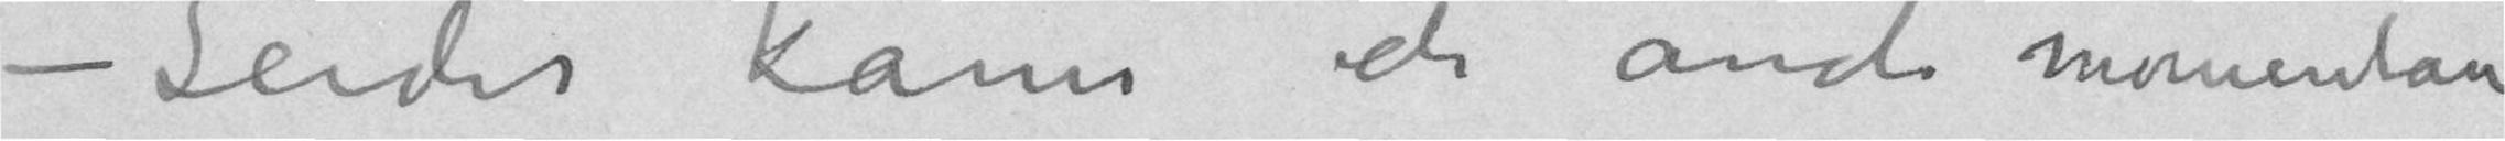– Leider kann ich auch momentan
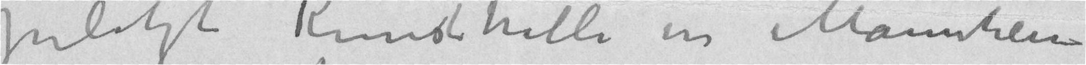zuletzt Kunsthalle in Mannheim
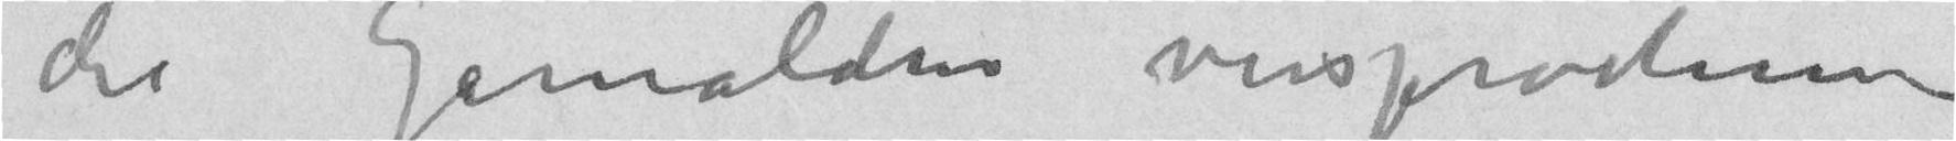die Gemälden versprochen
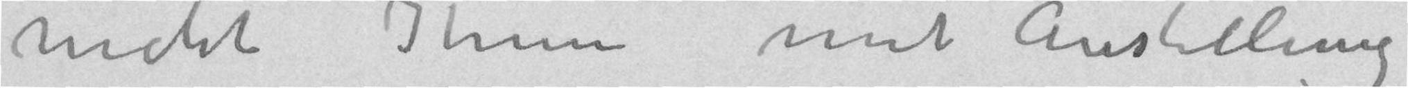nicht Ihnen mit Ausstellung
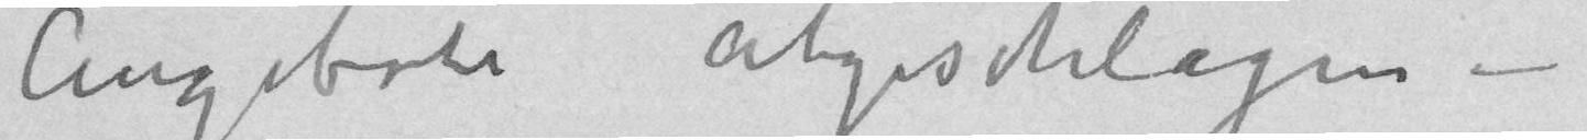Angebote abgeschlagen –
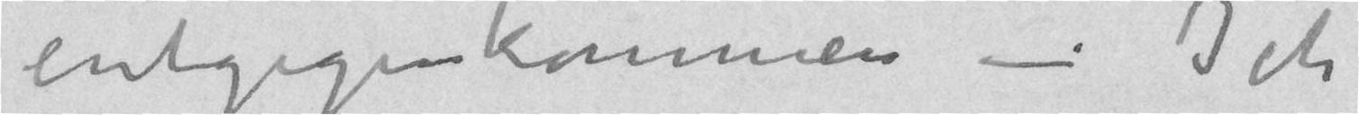entgegenkommen – Ich
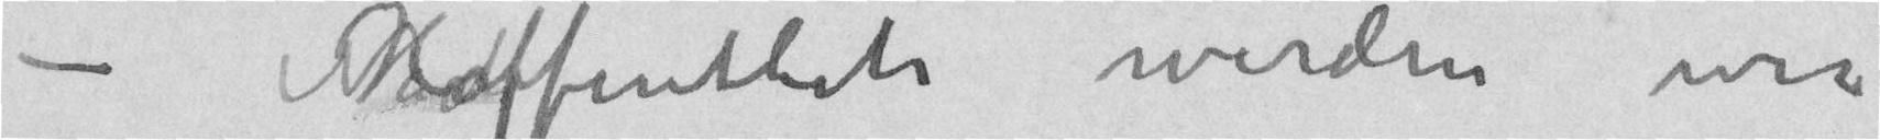– Hoffentlich werden wir
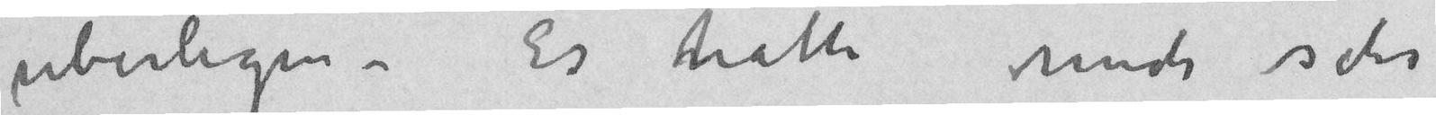uberlegen – Es hatte mich sehr
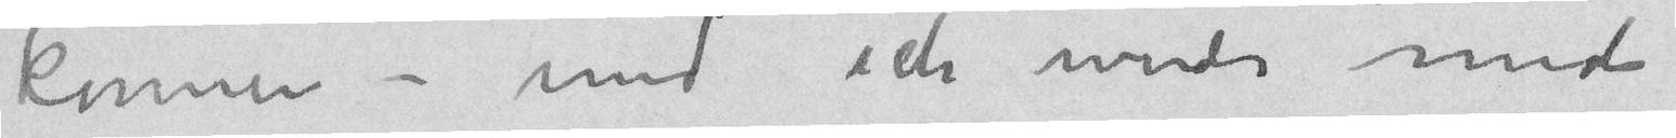können – und ich werde mich
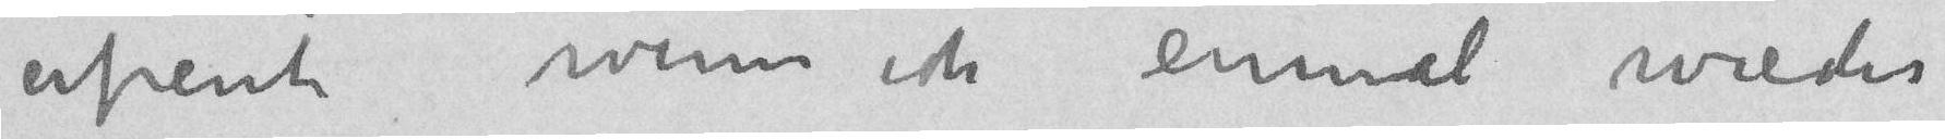erfreut wenn ich einmal wieder
